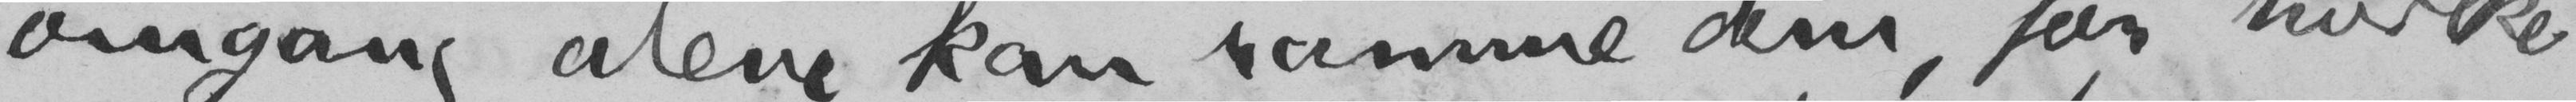omgang alene kan ramme dem, for hvilke
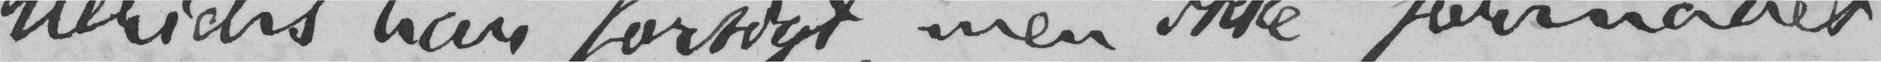Ulrichs har forsøgt, men ikke formaaet
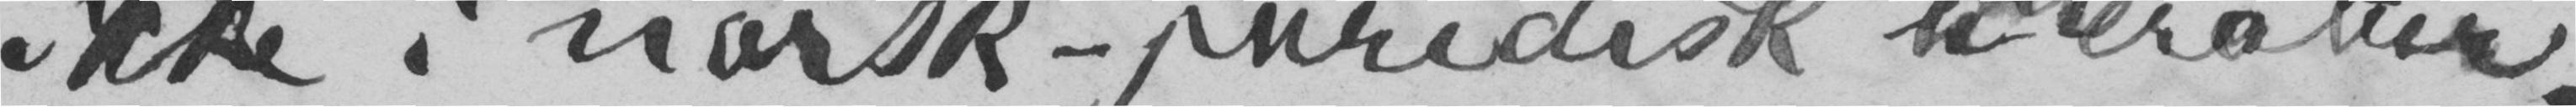ikke i norsk-juridisk literatur.
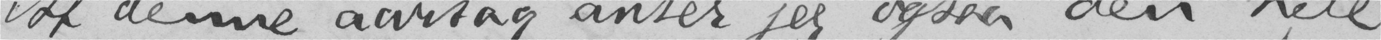Af denne aarsag anser jeg ogsaa den hele
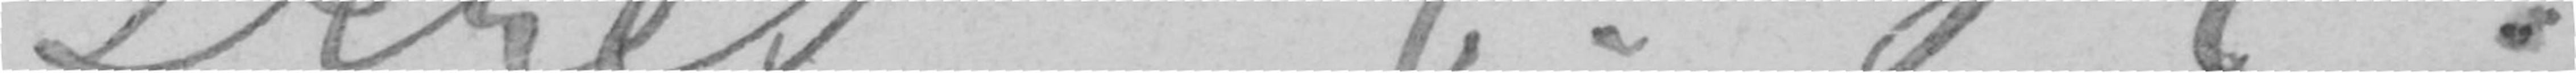Deres E. H.
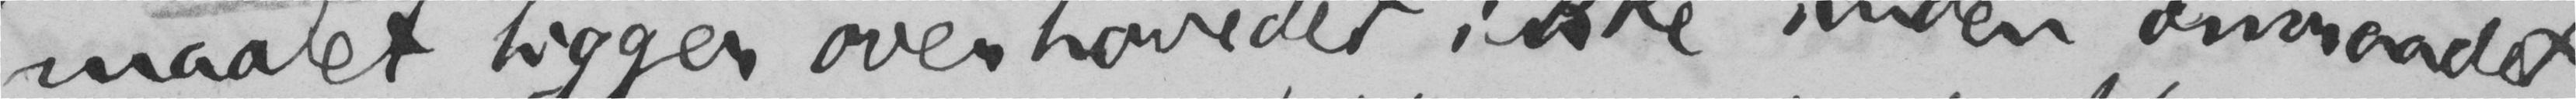maalet ligger overhovedet ikke inden omraadet
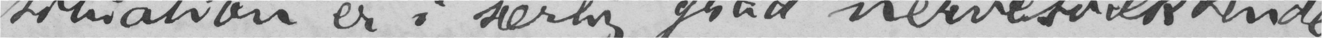situation er i særlig grad nervesvækkende.
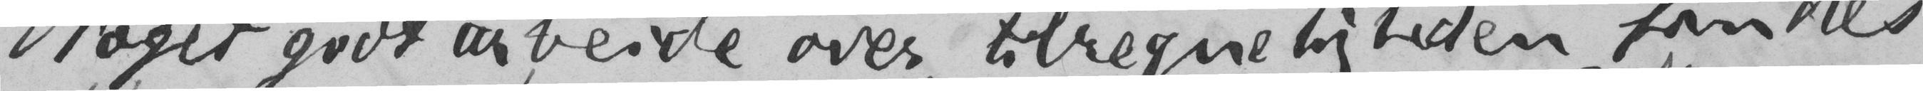Noget godt arbeide over tilregneligheden findes
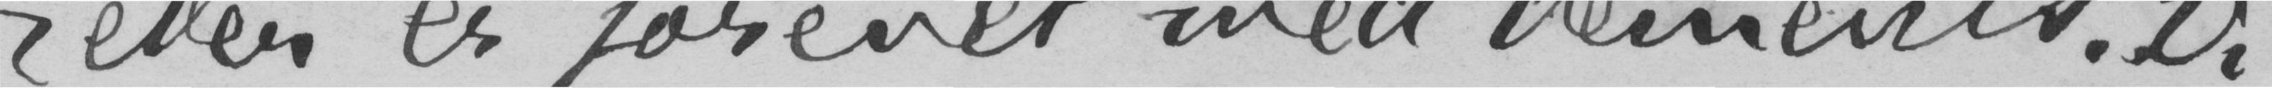eller er forenet med dements. Vi
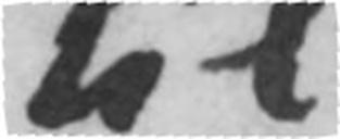til
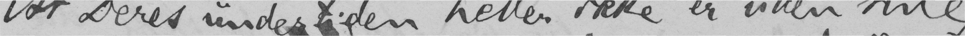At Deres undertiden heller ikke er uden sine
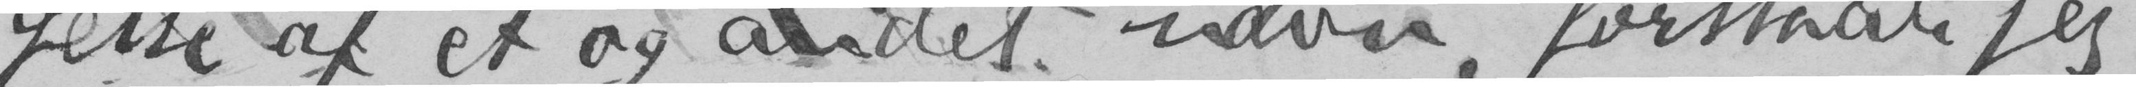gelse af et og andet navn, forstaar jeg
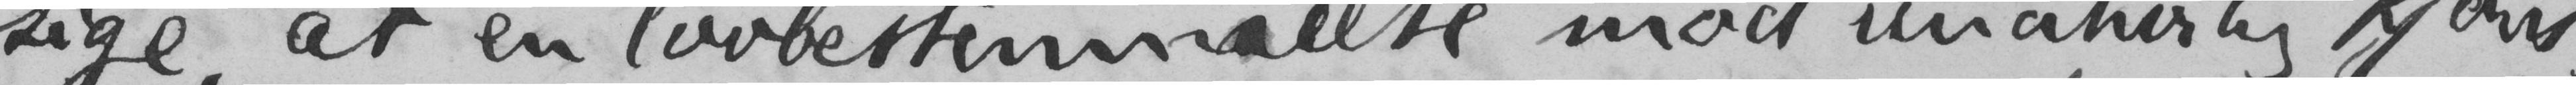sige, at en lovbestemmelse mod unaturlig kjøns-
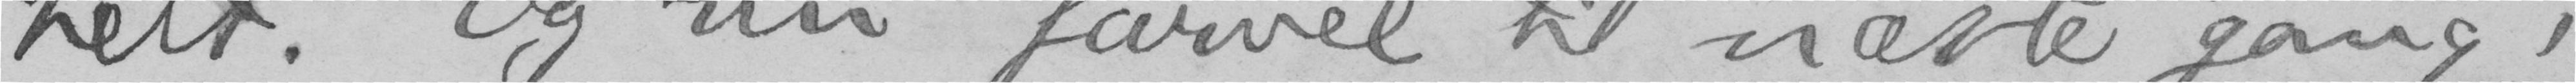helt. Og nu farvel til næste gang!
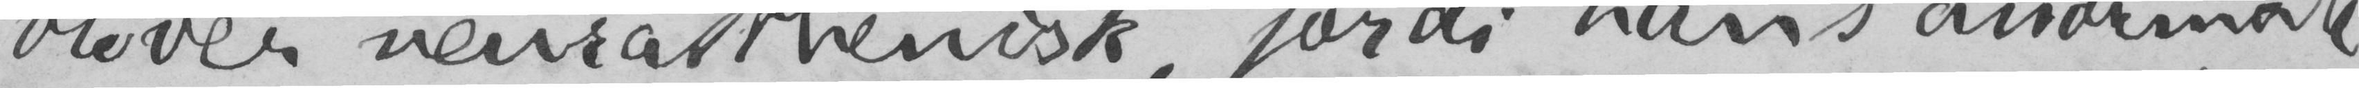bliver neurasthenisk, fordi hans anormale
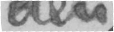den
